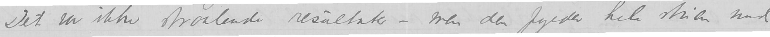Det var ikke straalende resultater – men den fylder hele stuen med
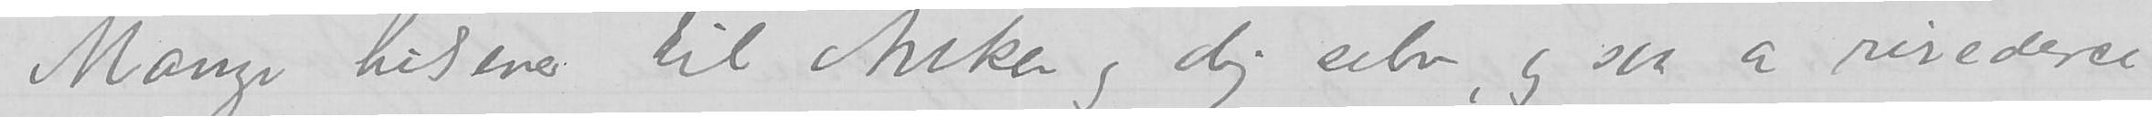Mange hilsener til Anker og dig selv, og saa a rivederci
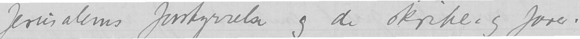Jerusalems forstyrrelse og de skriker og farer.
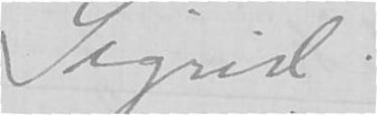Sigrid
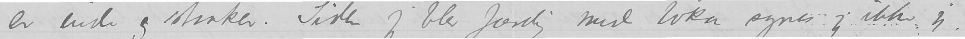er inde og staaker. Siden jeg blev færdig med boka synes jeg ikke jeg
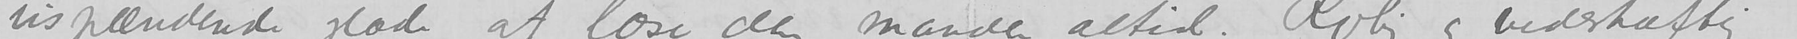uspændende glæde at læse den manden altid. Rolig og vedskæftig
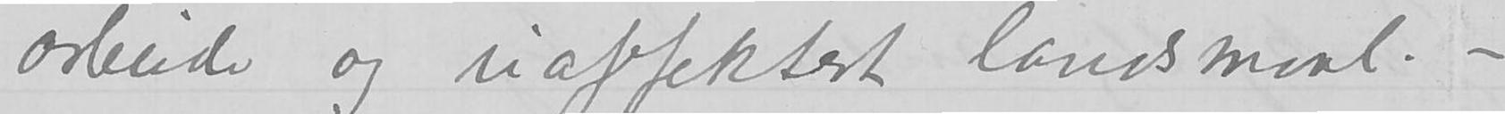arbeide og uaffektert landsmaal.-
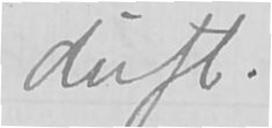duft.
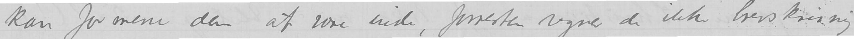kan formene dem at være inde, forresten regner de ikke brevskriving
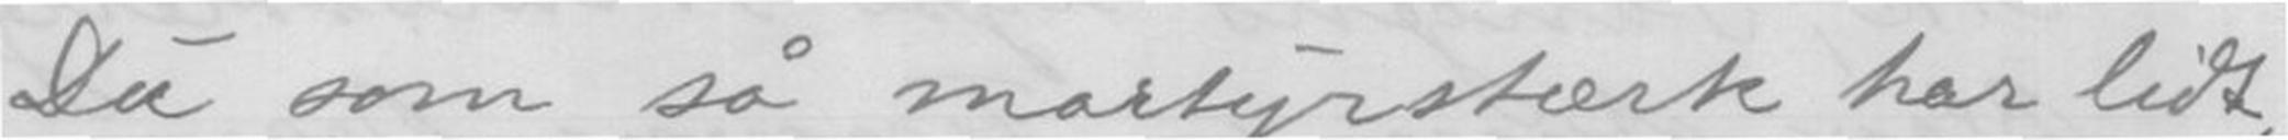Du som så martyrstærk har lidt
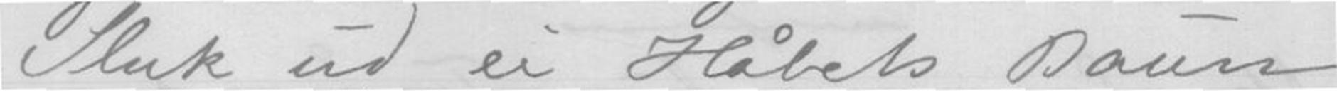Sluk ud ei Håbets Baun
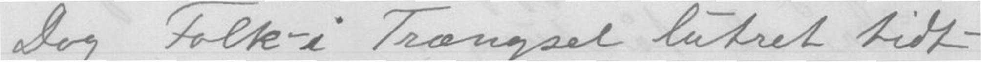Dog Folk-i Trængsel lutret tidt -
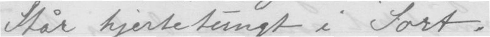Står hjertetungt i Sort.
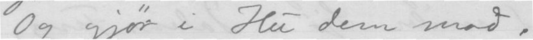Og gjør i Hu dem mod.
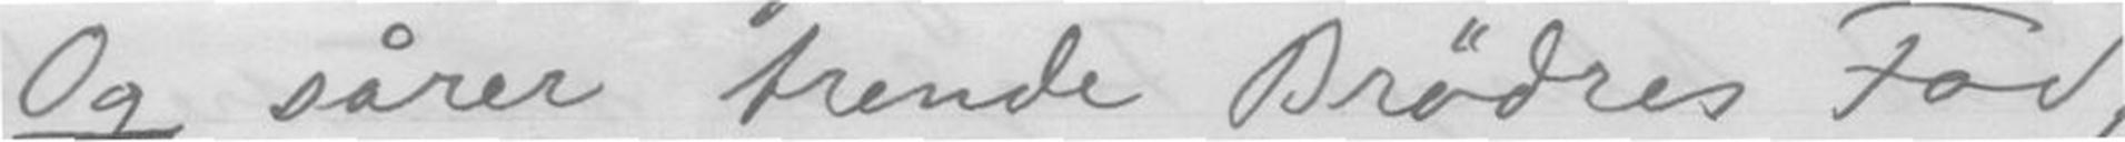Og sårer trende Brødres Fod,
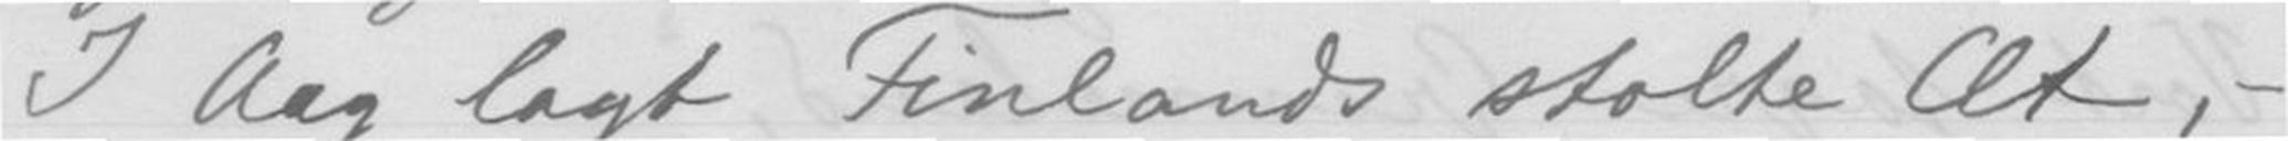I Aag lagt Finlands stolte Æt, -
